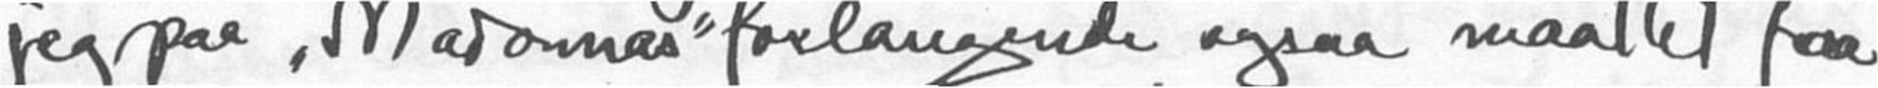jeg "Madonnas" forlangende ogsaa maattet faa
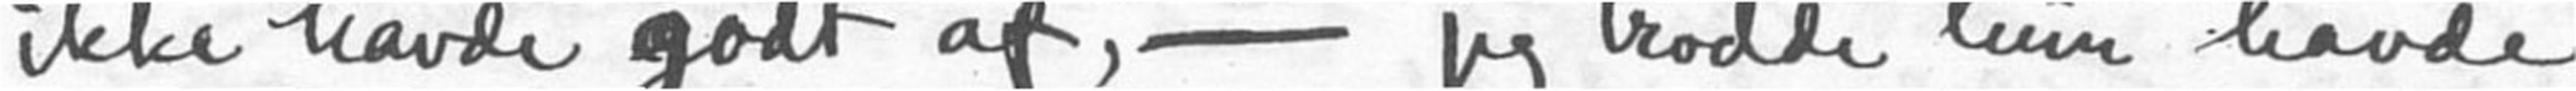ikke havde godt af, - jeg trodde hun havde
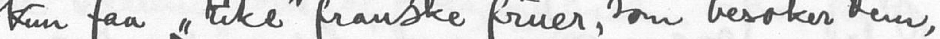kun faa "rike" franske fruer, som besøker dem,
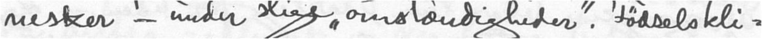nesker - under slige "omstændigheder". Fødselskli-
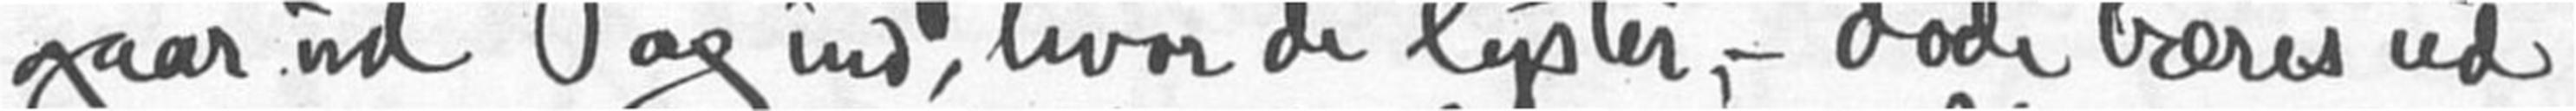gaar ud og ind, hvor de lyster, - døde bæres ud
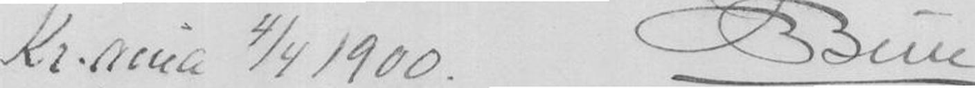Kr.ania 4/4 1900 JBBull
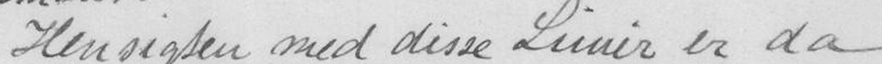Hesigten med disse Linier er da
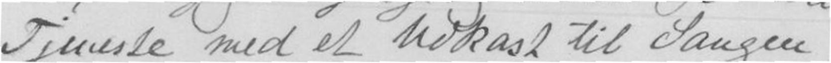Tjeneste med et Udkast til Sangen,
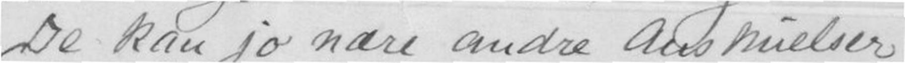De kan jo nære andre Anskuelser
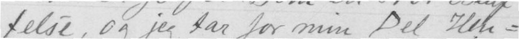felse, og jeg tar for min Del Hen-
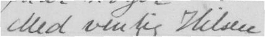Med venlig Hilsen
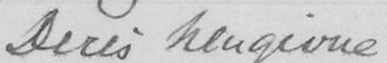Deres hengivne
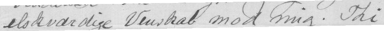elskværdige Venskab mod mig. Thi
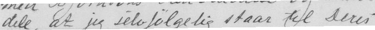dele, at selvfølgelig staar al Deres
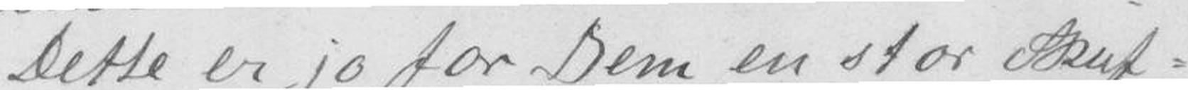Dette er jo for Dem en stor Skuf-
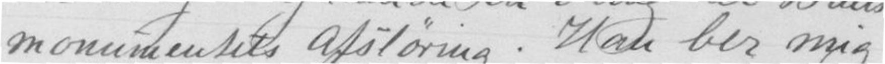monumentets Afsløring. Han ber mig
